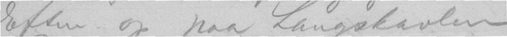Eftm. op paa Langskavlen
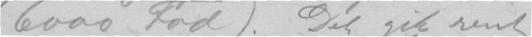(6000 Fod). Det gik rent
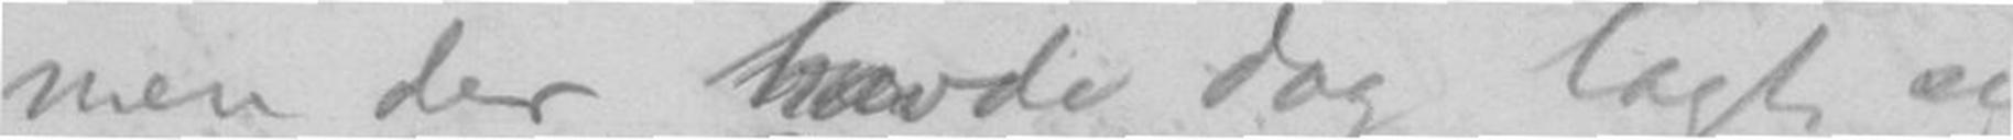men der havde dog lagt sig
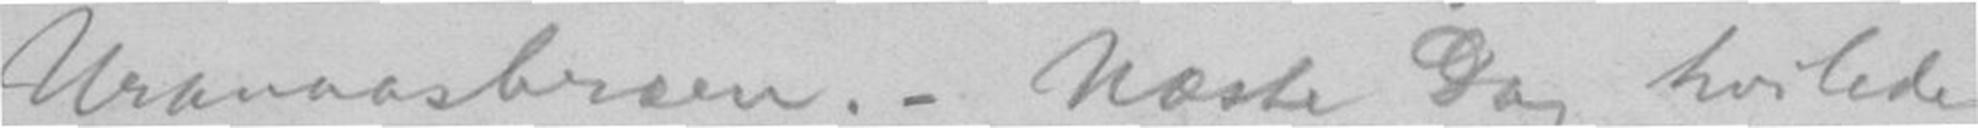Uranaasbræen. - Næste Dag hvilede
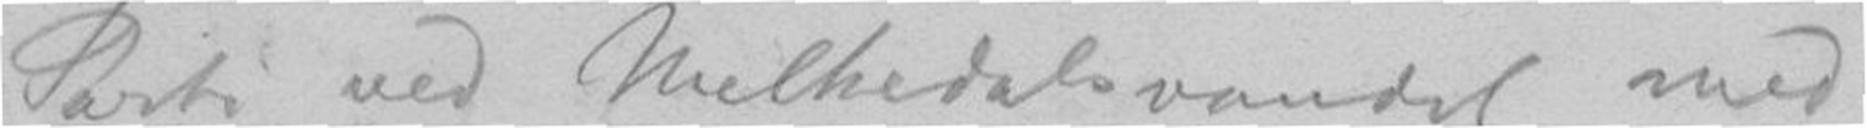Parti ved Melkedalsvandet med
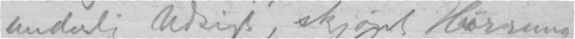underlig Udsigt, skjønt Hurrung-
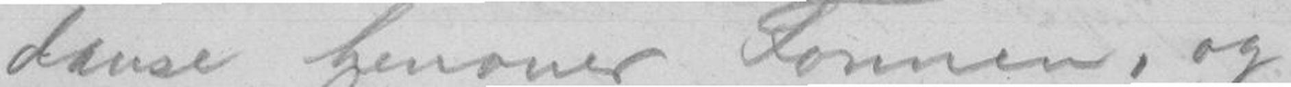danse henover Fonnen, og
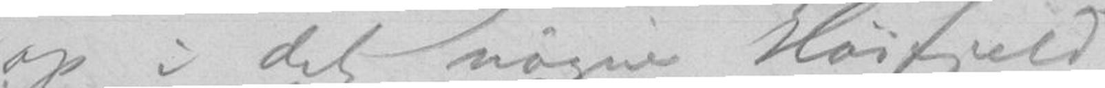op i det nøgne Høifjeld
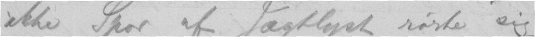ikke Spor af Jagtlyst rørte sig
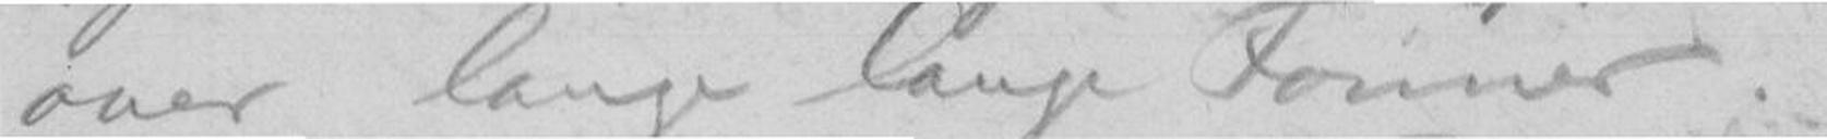over lange lange Fonner.
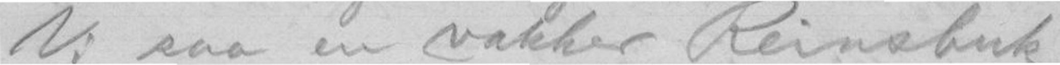Vi saa en vakker Reinsbuk
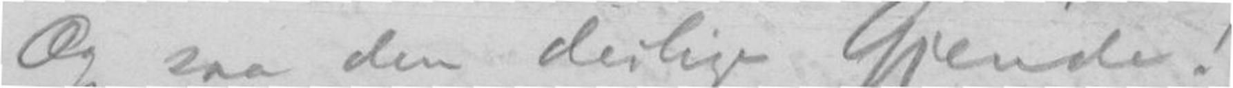Og saa den deilige Gjende!
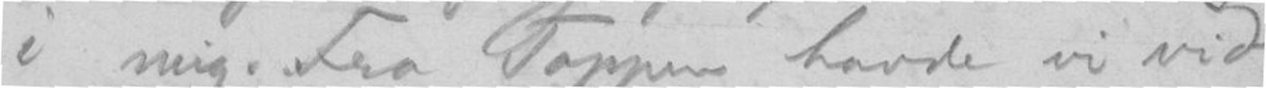i mig. Fra Toppen havde vi vid-
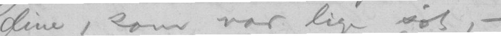dine, som var lige søt, -
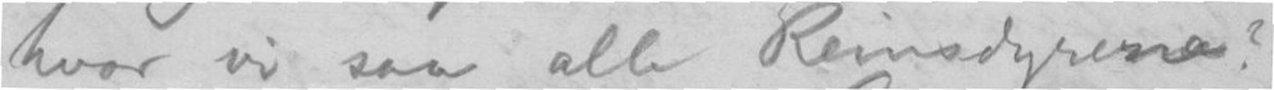hvor vi saa alle Reinsdyrene?
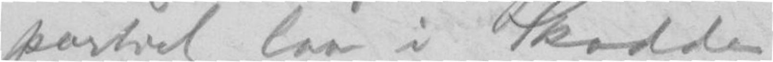partiet laa i Skodde.
